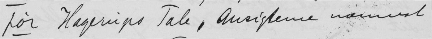før Hagerups Tale, Ansigterne nærmest
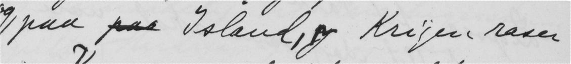paa  Island, og Krigen raser
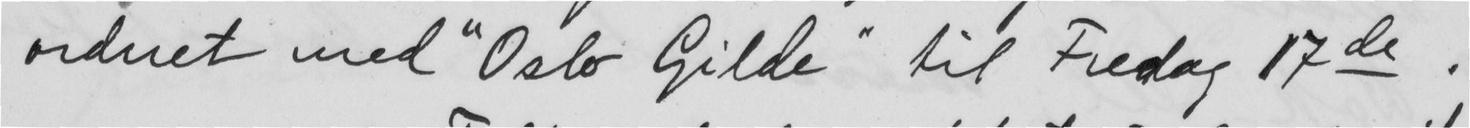ordnet med "Oslo Gilde" til Fredag 17de.
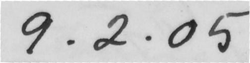9.2.05
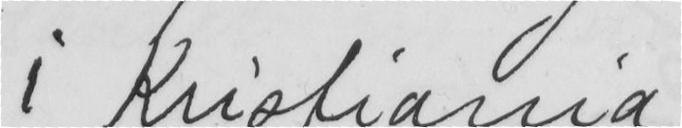i Kristiania
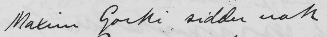Maxin Gorki sidder nok
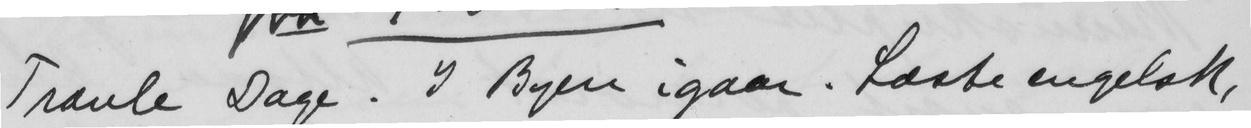Travle Dage. I Byen igaar. Læste engelsk,
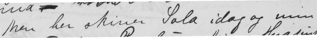Men her skiner Sola idag og min
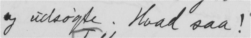og udsøgte. Hvad saa!
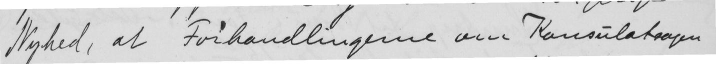Nyhed, at Forhandlingerne om Konsulatsagen
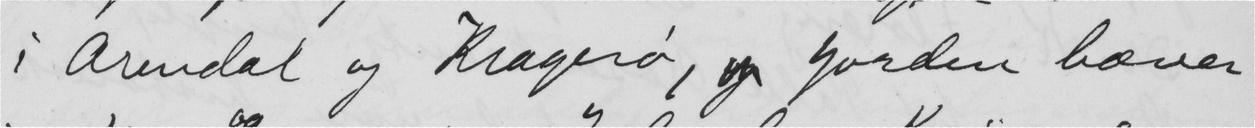i Arendal og Kragerø,  Jorden bæver
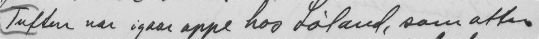Tuften var igaar oppe hos Løland, som atter
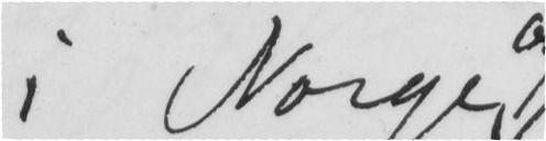i Norge
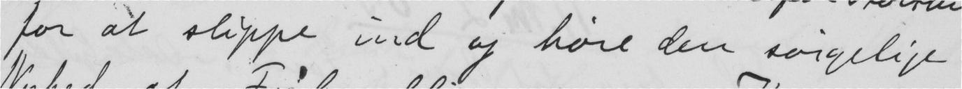for at slippe ind og høre den sørgelige
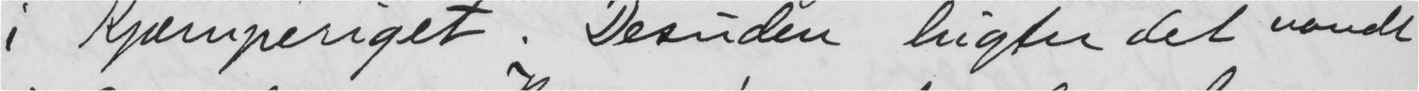i kjæmperiget. Desuden lugter det vondt
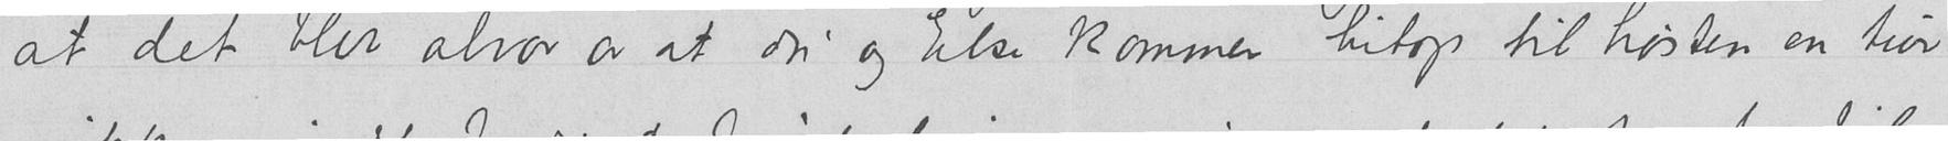at det blir alvor av at du og Else kommer hitop til høsten en tur
